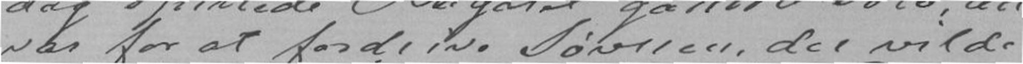var for at fordrive Søvnen der vilde
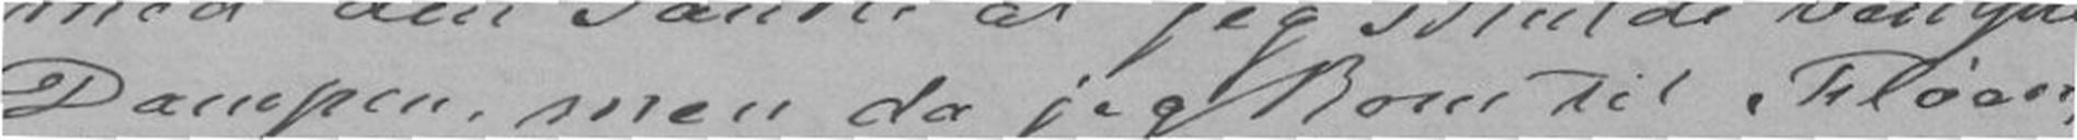Dampen, men da jeg kom til Fløen,
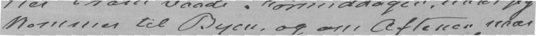kommer til Byen, og om Aftenen naar
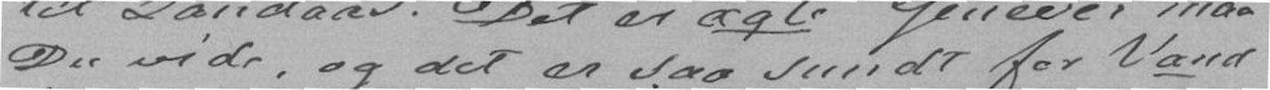Du vide, og det er saa smukt for Vand
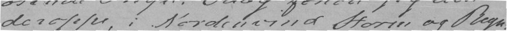deroppe, i Nordenvind Storm og Regn,
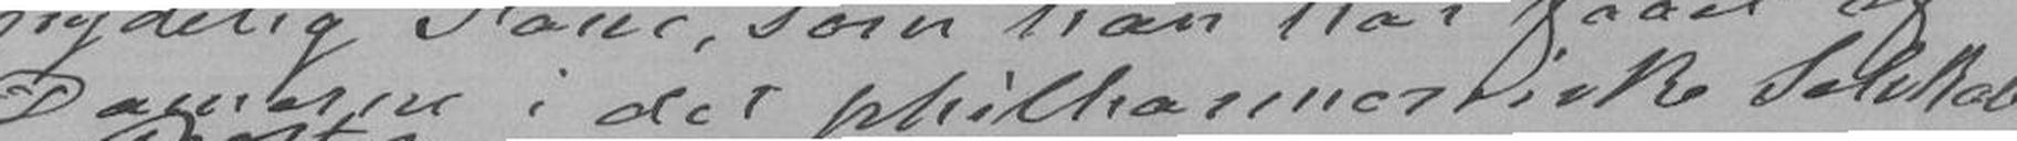Damerne i det philharmoniske Selskab
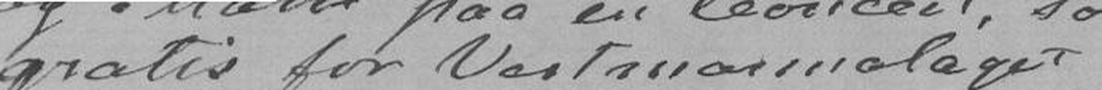gratis for Vestmannalaget
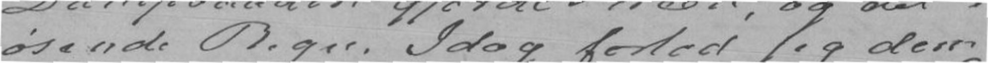øsende Regn. Idag forlod jeg dem
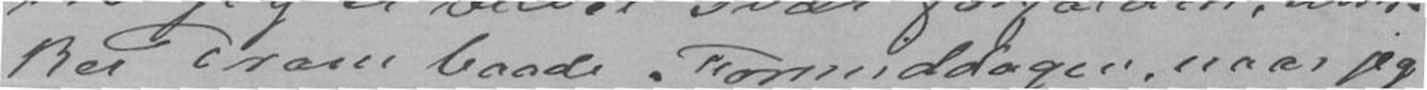ker Dram baade Formiddagen, naar jeg
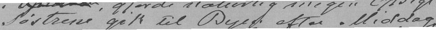Søstrene gik til Byen efter Middag
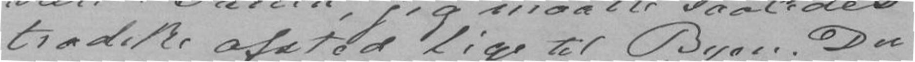tradske afsted lige til Byen. Du maa
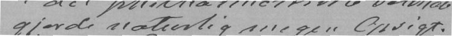gjorde naturlig megen Opsigt.
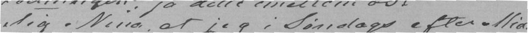Sig Nina at jeg i Søndags efter Mid-
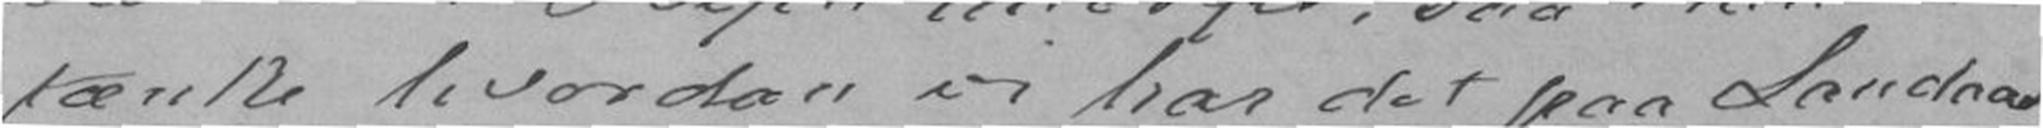tænke hvordan vi har det paa Landaas.
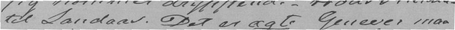til Landaas. Det er ægte Genever, maa
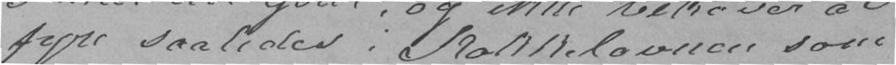fyre saaledes i Kakkelovnen som
